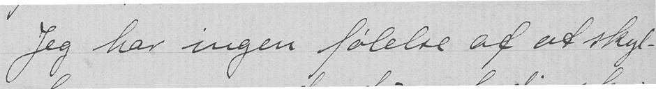Jeg har ingen følelse af at skyl-
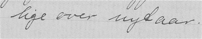lige over nytaar.
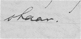staar. -
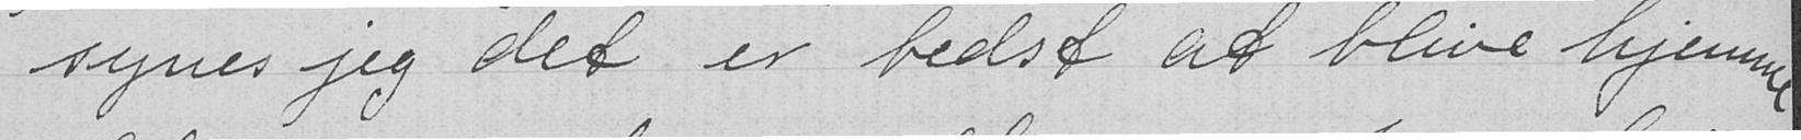synes jeg det er bedst at blive hjemme
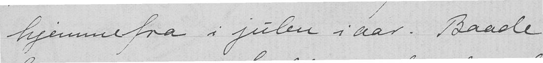hjemmefra i julen i aar. Baade
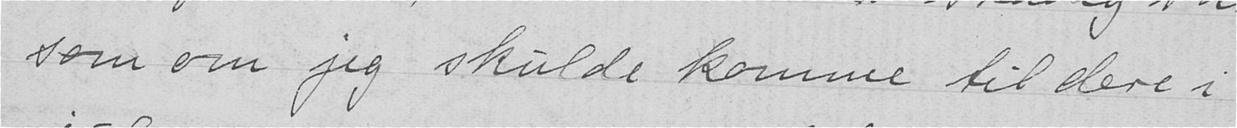som om jeg skulde komme til dere i
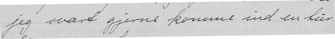jeg svært gjerne komme ind en tur
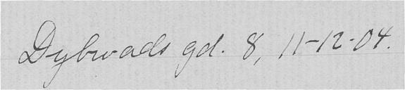Dybvads gd. 8, 11-12-04.
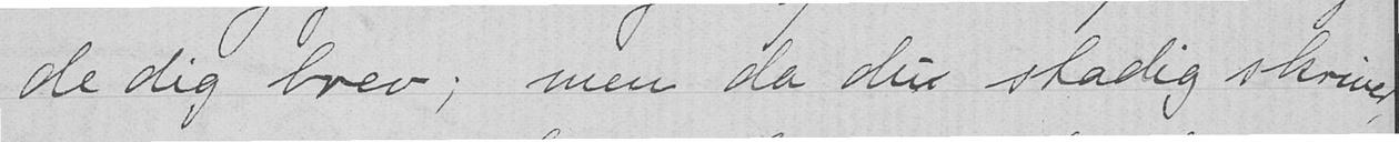de dig brev; men da du stadig skriver,
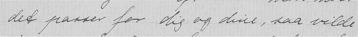det passer for dig og dine, saa vilde
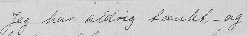Jeg har aldrig tænkt, - og
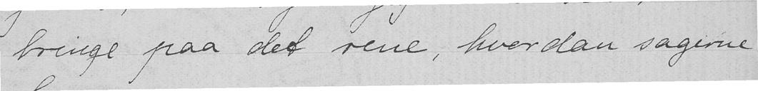bringe paa det rene, hvordan sagerne
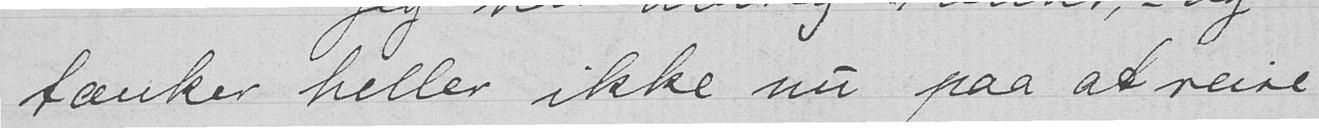tænker heller ikke nu paa at reise
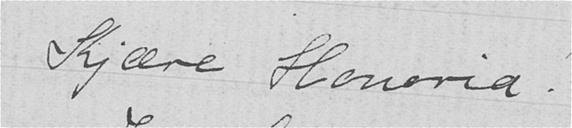Kjære Honoria!
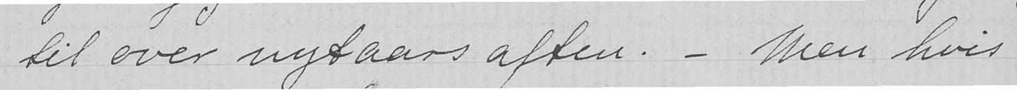til over nytaars aften. - Men hvis
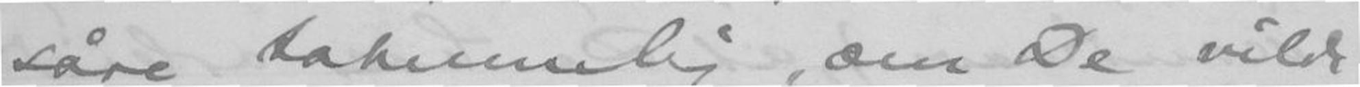såre taknemlig om De vilde
